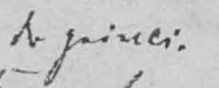de Princi-
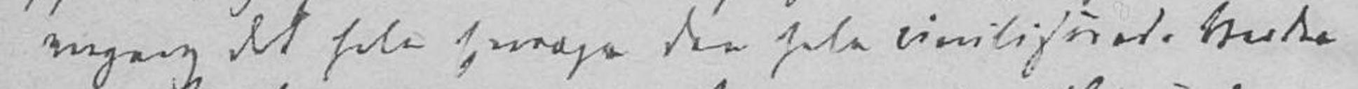engang det hele Europa den hele civiliserede Verden
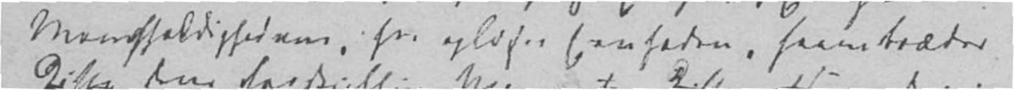Mandfoldighedens, her opløses Eenheden, fremtræder
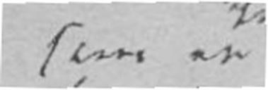som en
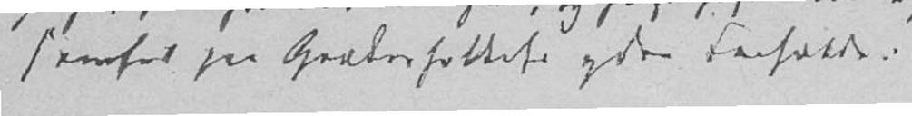somhed paa Grækerfolkets ydre Forholde.
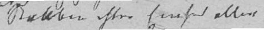Stræben efter Eenhed eller
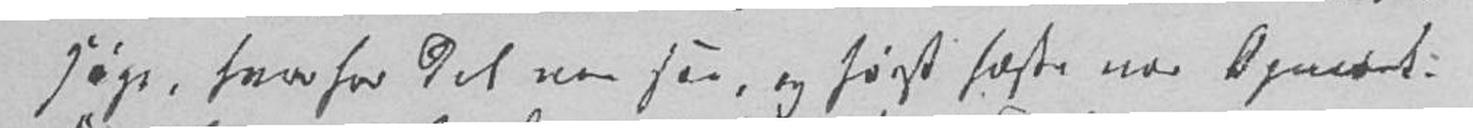søge, hvorfor det var saa, og først fæste vor Opmærk-
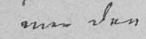over den
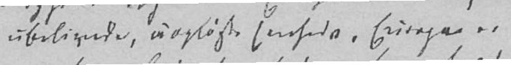ubelivede, uopløste Eenheds. Europa er
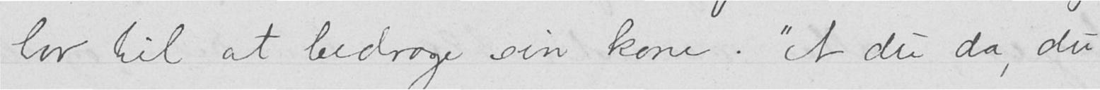lov til at bedrage sin kone. "A du da, du
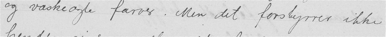og vaskeægte farver. Men det forstyrrer ikke
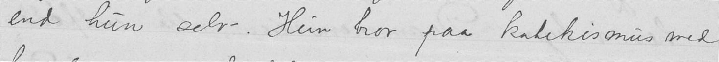end hun selv. Hun bor paa katekismus med
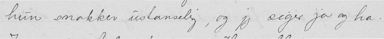hun snakker ustanselig, og jeg siger ja og ha.
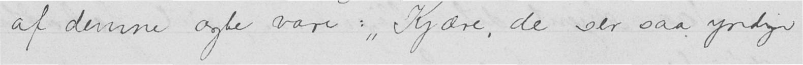af denne ægte vare: "Kjære, de ser saa yndige
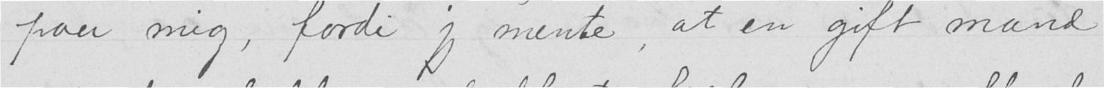paa mig, fordi jeg mente, at en gift mand
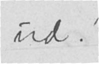ud."
